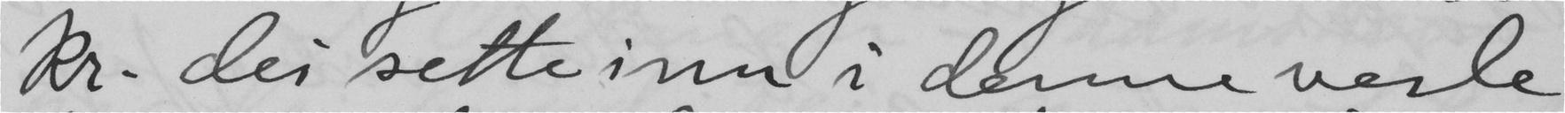kr. dei sette inn i denne vesle
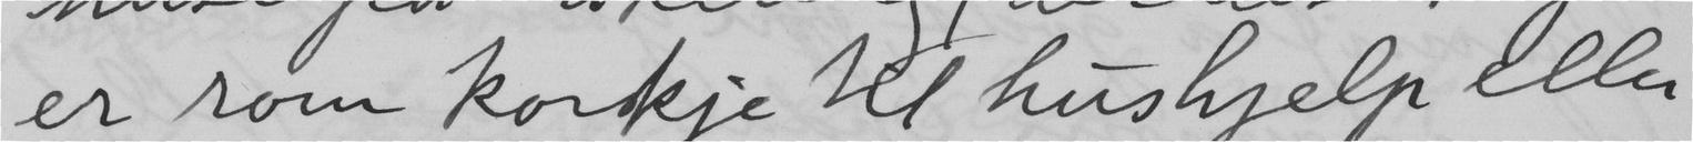er som korkje til hushjelp eller
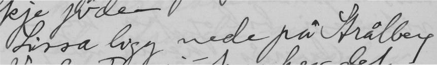Lissa ligg nede på Strålberg
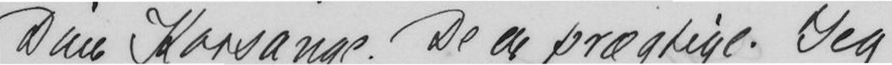Dine Korsange. De er prægtige. jeg
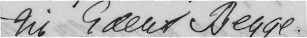til Edert Begge.
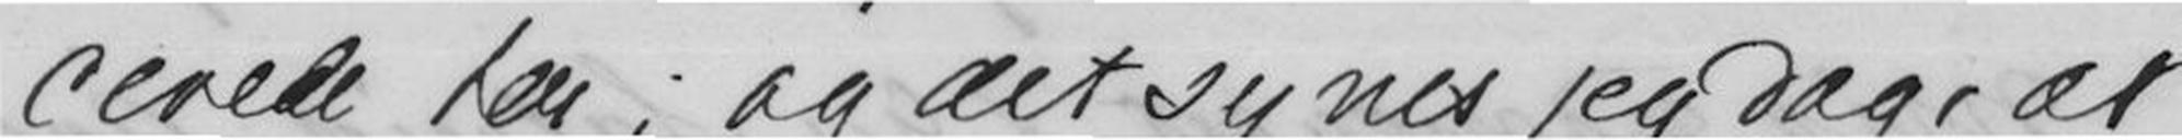cerede her; og det synes jeg dog at
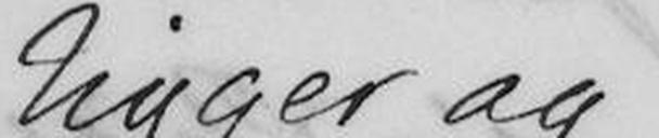ligger og
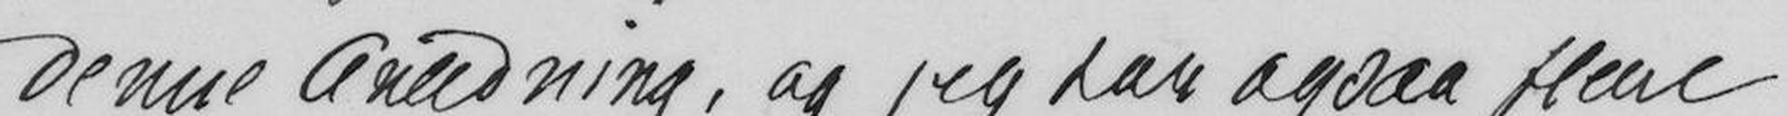denne Anledning, at jeg ogsaa flere
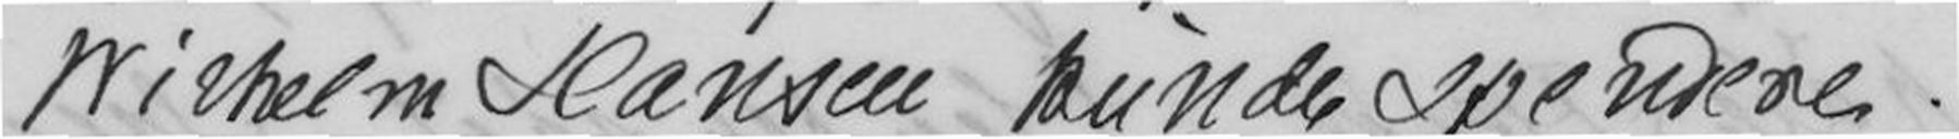Wilhelm Hansen kunde spendere.
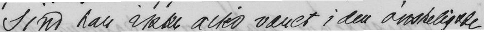Sind har ikke altid været i den ønskeligste
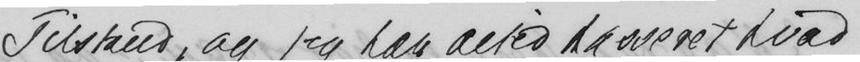Tilstand, og jeg har altid kasseret hvad
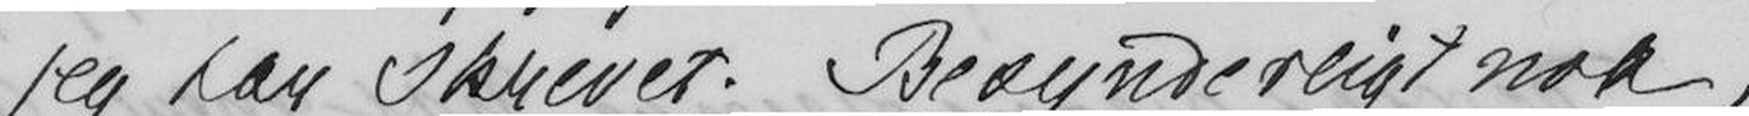jeg har skrevet. Besynderligt nok,
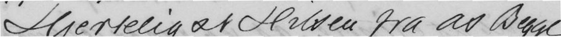Hjerteligst Hilsen fra os Begge
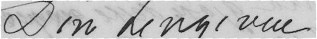Din hengivne
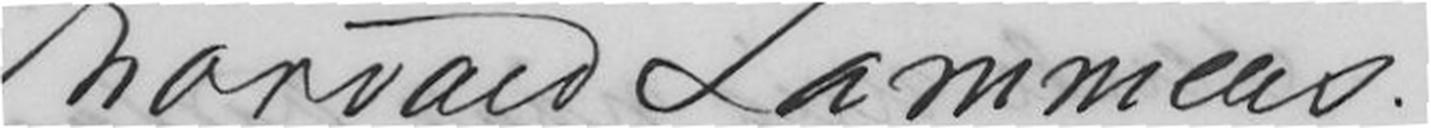Thorvald Lammers
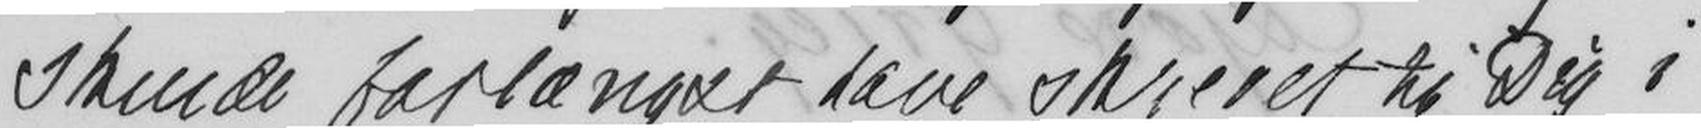skulde forlængst have skrevet til Dig i
